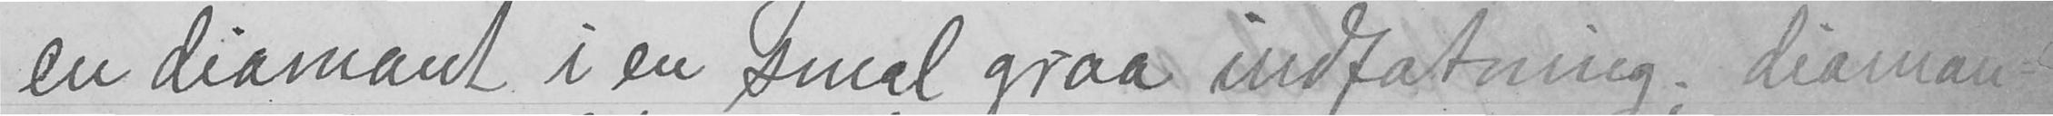en diamant i en smal graa indfatning; diaman-
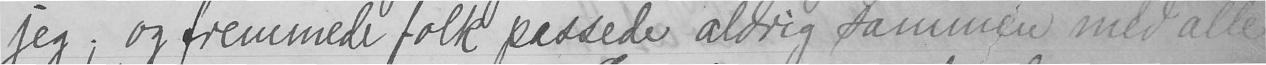jeg; og fremmede folk paasede aldrig sammen med alle
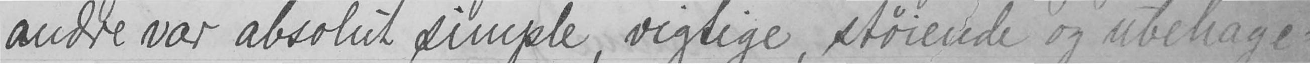andre var absolut simple, vigtige, støiende og ubehage-
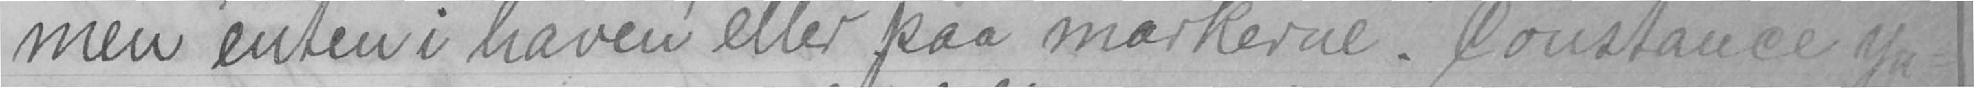men enten i haven eller paa markerne. Constance yn-
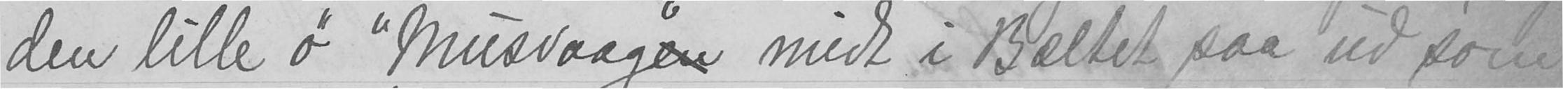den lille ø "Musvaag"en midt i Bæltet saa ud som
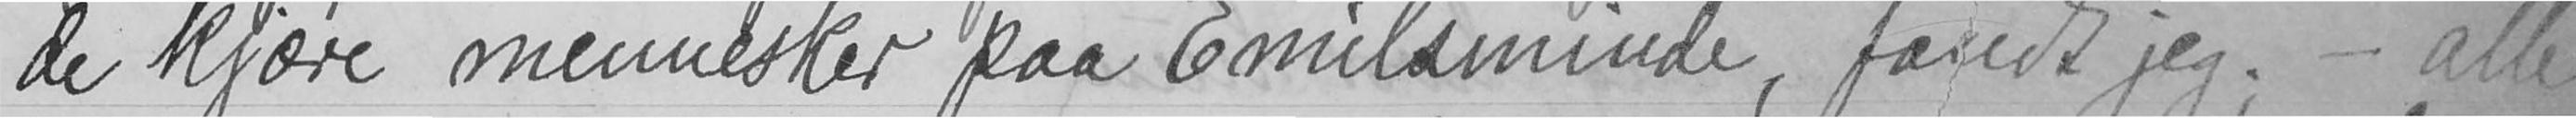de kjære mennesker paa Emilsminde, fandt jeg; - alle
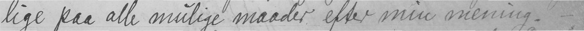lige paa alle mulige maader efter min mening. -
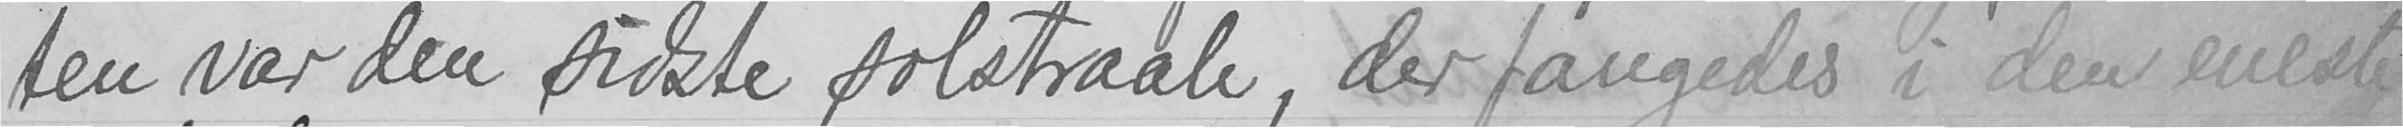ten var den sidste solstraale, der fangedes i den eneste
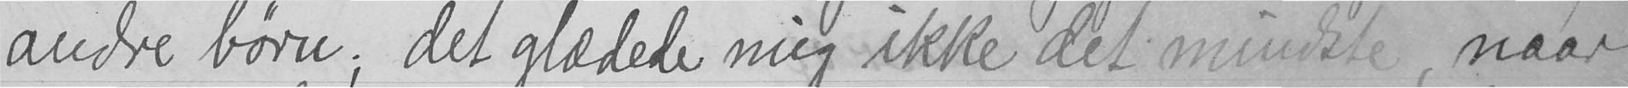andre børn; det glædede mig ikke det mindste, naar
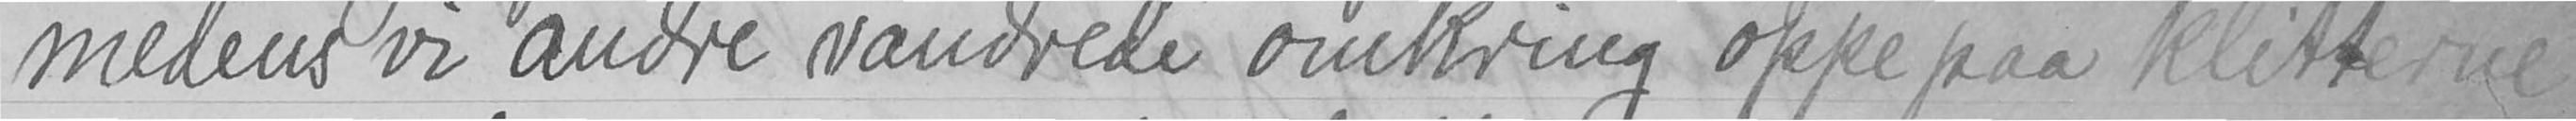medens vi andre vandrede omkring oppe paa klitterne
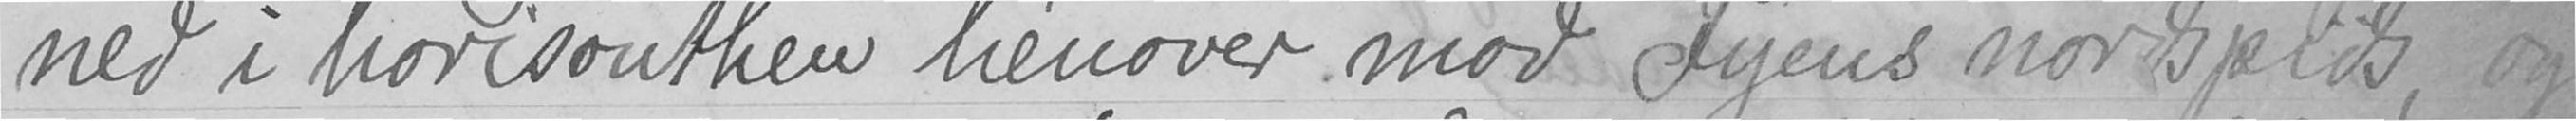ned i horisonthen henover mod Fyens nordspids, og
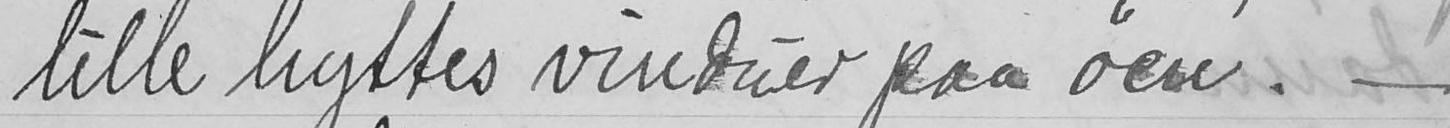lille hyttes vinduer paa øen. -
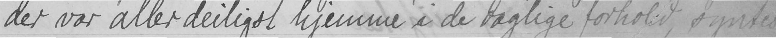der var aller deiligst hjemme i de daglige forhold, syntes
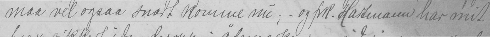maa vel ogsaa snart komme nu; - og frk. Hartmann har mit
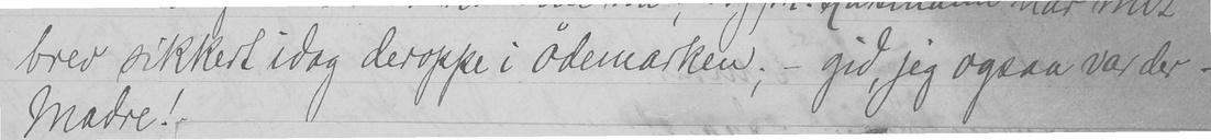brev sikkert idag deroppe i ødemarken; - gid jeg ogsaa var der der -
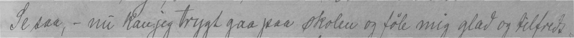Se saa, - nu kan jeg trygt gaa paa skolen og føle mig glad og tilfreds
